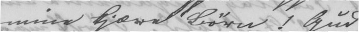mine kjære børn! Gud
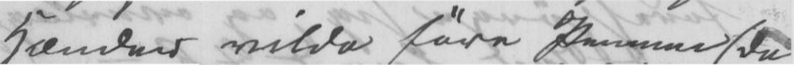hænder vilde føre Pennen (Du
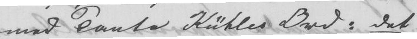med Tante Kühles Ord: det
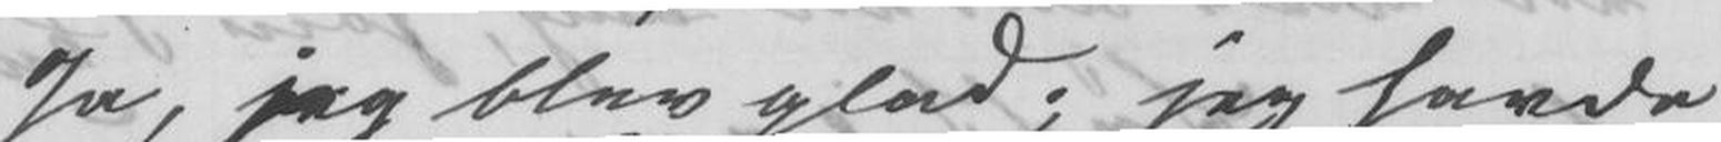Ja, jeg blev glad; jeg havde
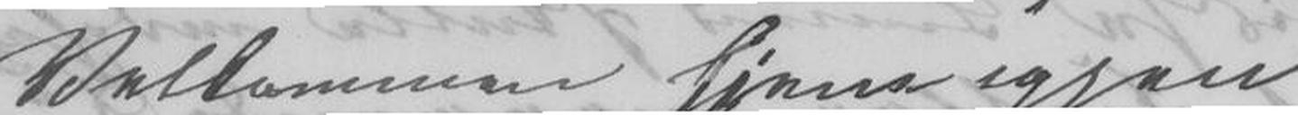Velkommem hjem igjen,
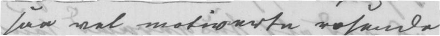saa vel motiverte rosende
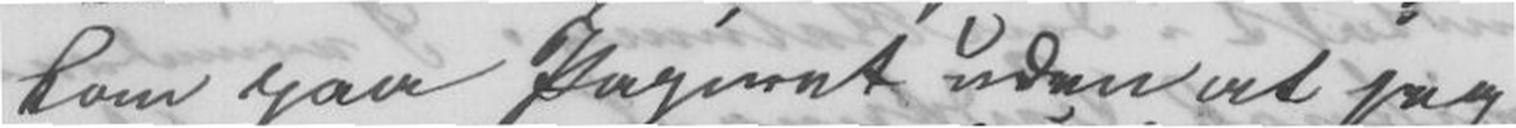kom paa papiret uden at jeg
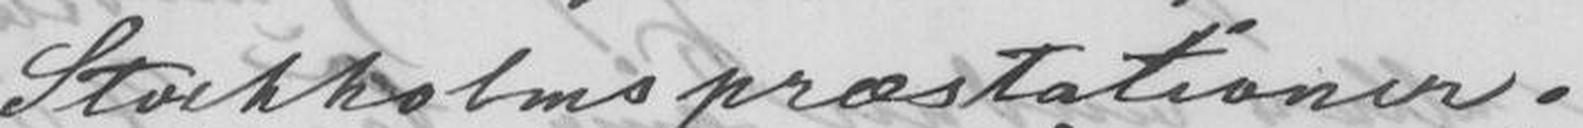Stockholmspræstationer.
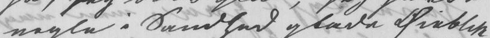nogle i Sandhed glade Øieblik
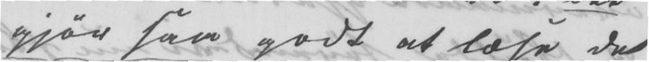gjør saa godt at læse Du
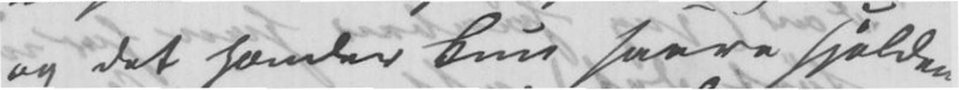og det samler kun saare sjelden
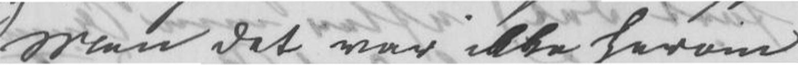Men det var ikke herom,
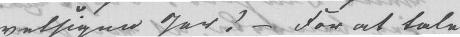velsigne Jer! - For at tale
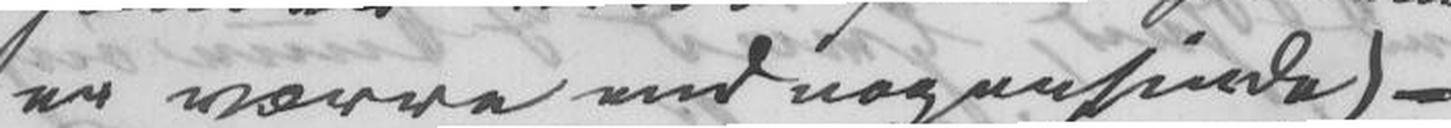er værre endnogensinde) -
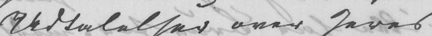Udtalelser over Jeres
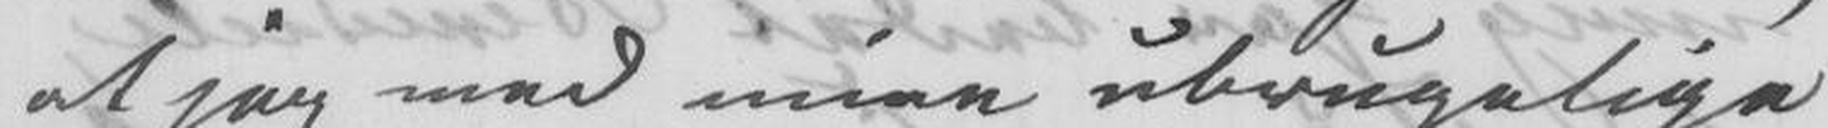at jeg med mine ubrugelige
